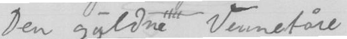Den gyldne Vennetåre
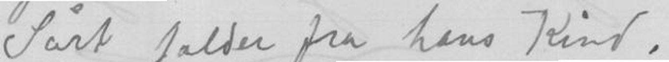Sårt falder fra hans Kind.
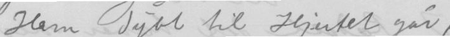Ham dybt til Hjertet går,
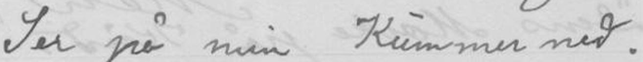Ser på min Kummer ned.
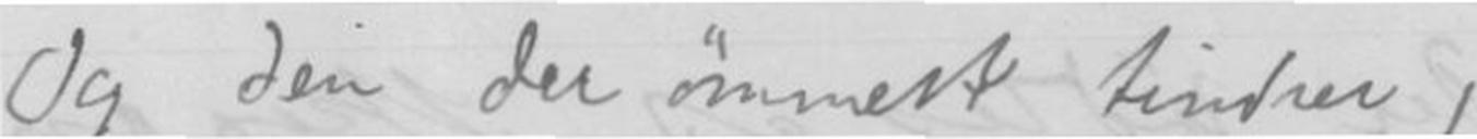Og den der ømmest tindrer,
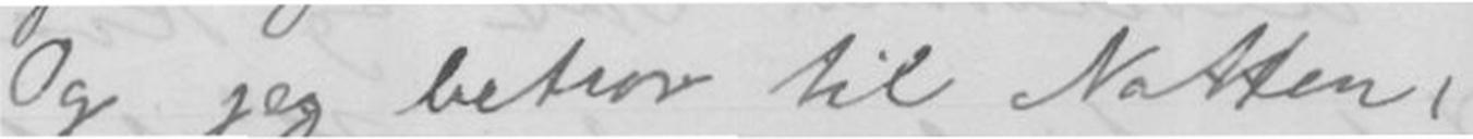Og jeg betror til Natten,
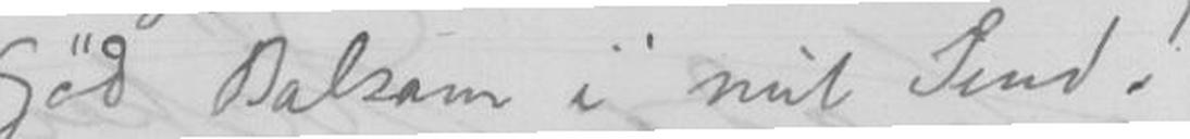Gød Balsam i mit Sind!
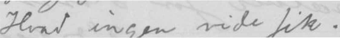Hvad ingen vide fik.
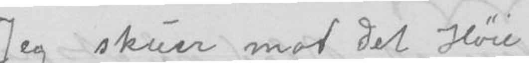Jeg skuer mod det Høie
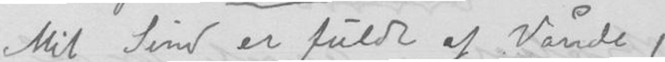Mit Sind er fuldt af Vånde,
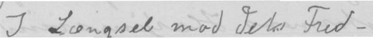I Længsel mod dets Fred -
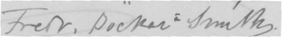Fredr. Döcker=Smith
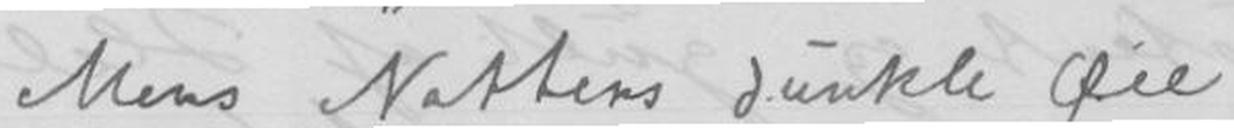Mens Natters dunkle Øie
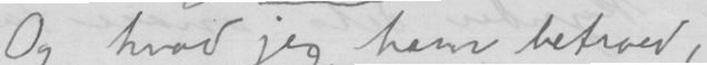Og hvad jeg ham betror,
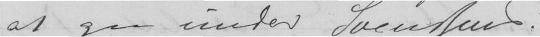at gaa under Svendsens
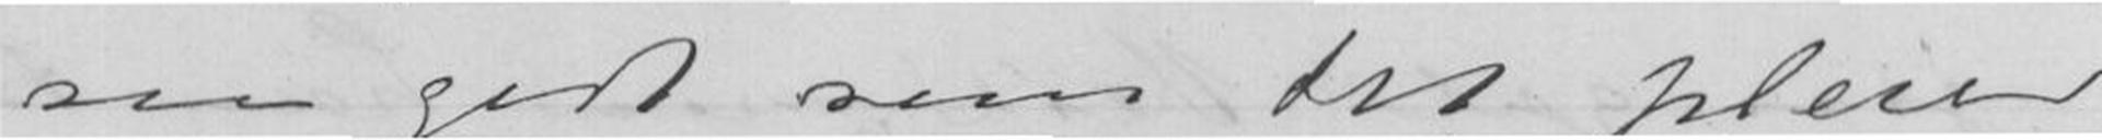saa godt som det pleier
